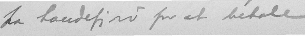fra Sandefjord for at betale
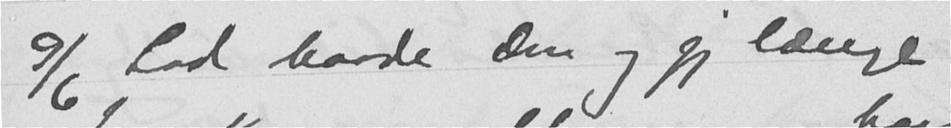9/6 Sad baade Don og jeg længe
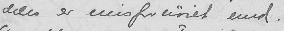deles er misfornøiet med.
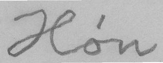Høn
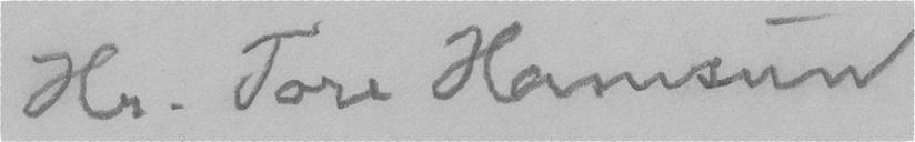Hr. Tore Hamsun
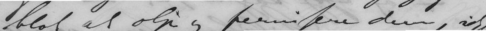blot at olje og fernisere dem, idet
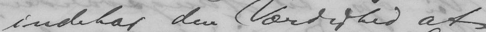indehar den Værdighed at
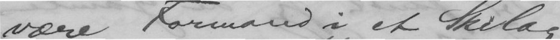være Formand i et Skilag,
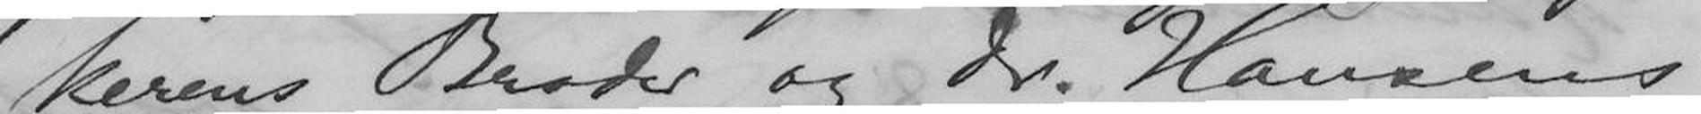kerens Broder og Dr. Hansens
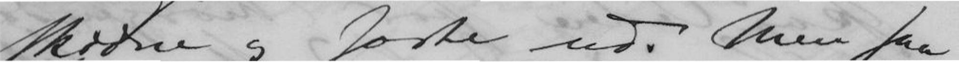skidne og sorte ud. Men saa
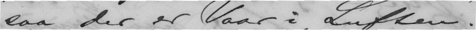saa der er Vaar i Luften.
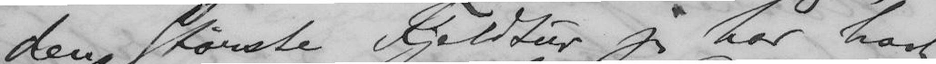den største Fjeldtur jeg har havt
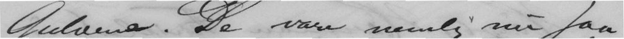Gulvene. De vare nemlig nu saa
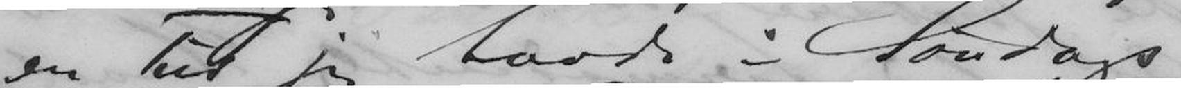en Tur jeg havde i Søndags,
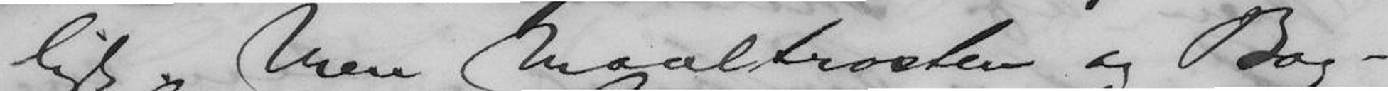ligt. Men Maaltrosten og Bog-
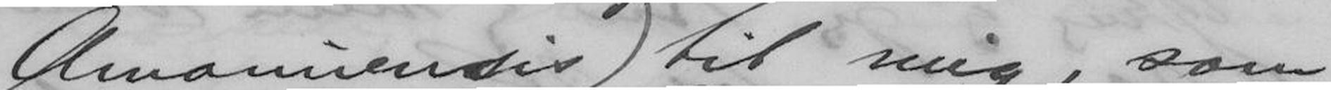Amanuensis) til mig, som
